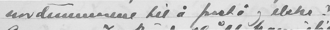nordmennene til å forstå og elske?
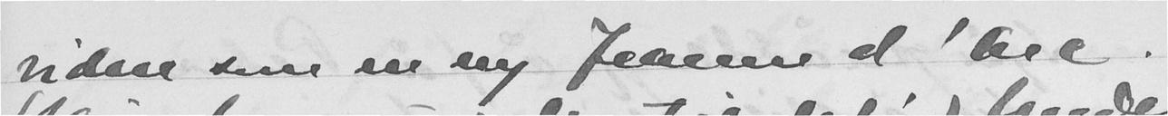vidne som en ny Jeanne d'arc.
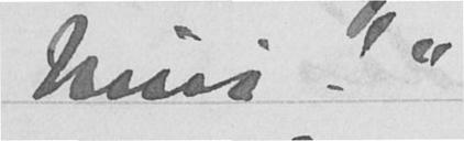Nini!"
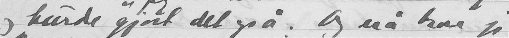og burde gjort det også. Og nå har jeg
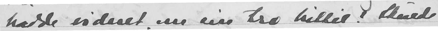hadde vidnet om sin tro hittil! Skulde
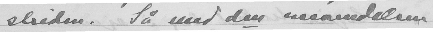striden. Så med den mandelsen
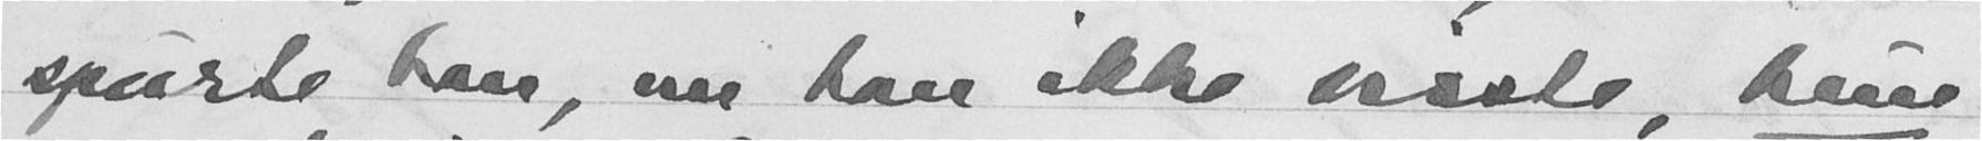spurte han, om han ikke visste, hun
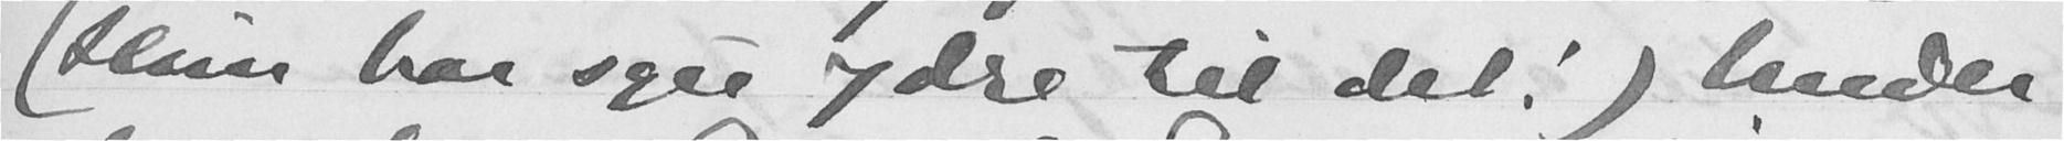(Hun har sgu ydre til det!) Under
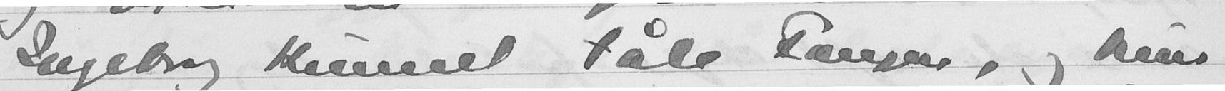Ingeborg kunnet tåle Fangen, og hun
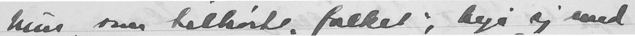hun, som tilhørte "folket", begi sig med
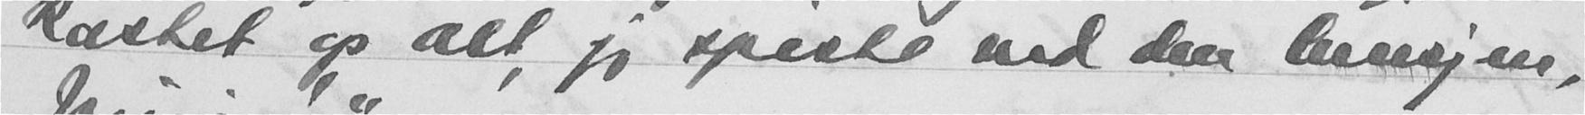kastet op alt, jeg spiste ved den lunsjen,
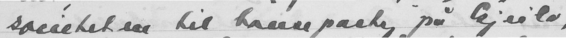societeten til houseparty på Geilo,
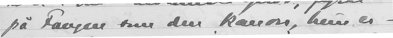på Fangen som den kanon, hun er -
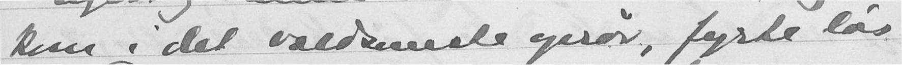kom i det voldsomste oprør, fyrte løs
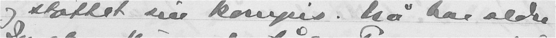og støttet sin kompis. Nå har aldri
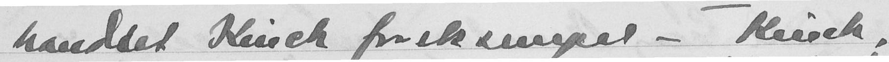handlet Kinck for eksempel - Kinck,
